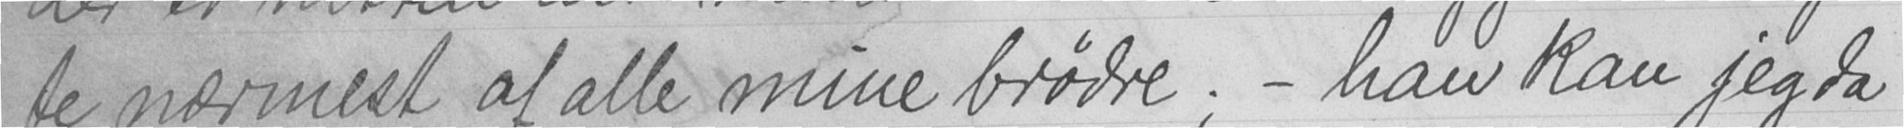te nærmest af alle mine brødre; - han kan jeg da
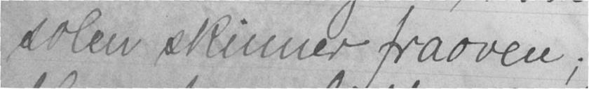solen skinner fraoven;
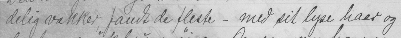delig vakker, fandt de fleste - med sit lyse haar og
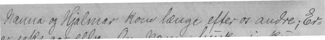Nanna og Hjalmar kom længe efter os andre; Er-
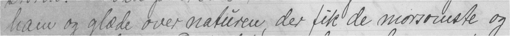ham og glæde over naturen, der fik de morsomste og
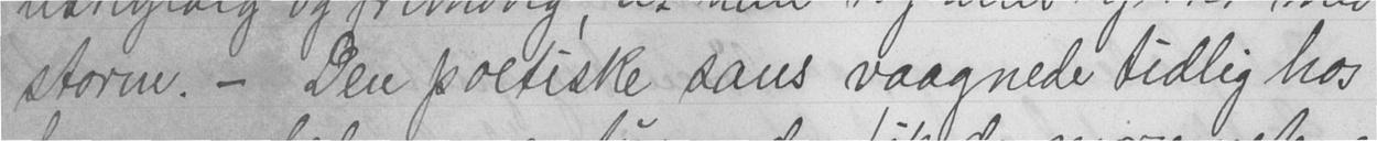storm. - Den poetiske sans vaagnede tidlig hos
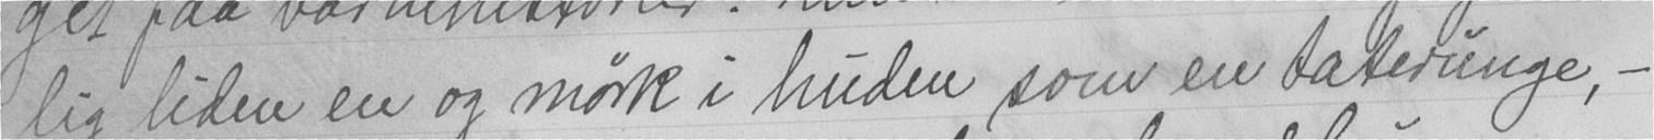lig liden en og mørk i huden som en taterunge, -
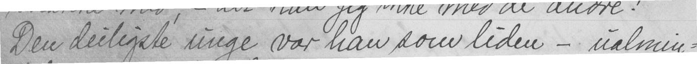Den deligste unge var han som liden - ualmin-
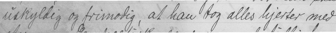uskyldig og frimodig, at han tog alles hjerter med
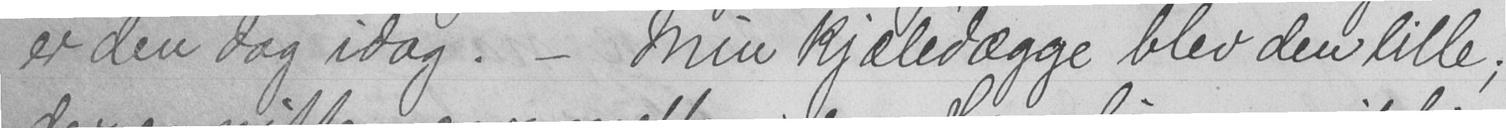er den dag idag. - Min kjæledægge blev den lille;
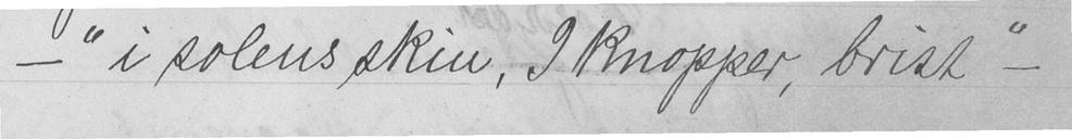- "i solens skin, I knopper, brist" -
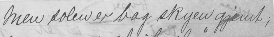Men solen er bag skyen gjemt;
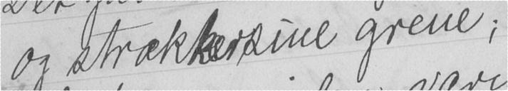og strækker sine grene;
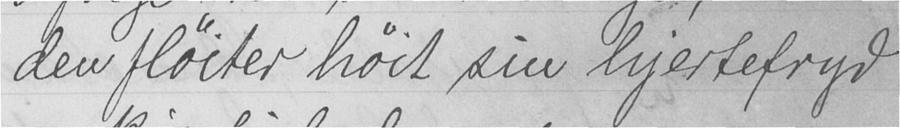den fløiter høit sin hjertefryd
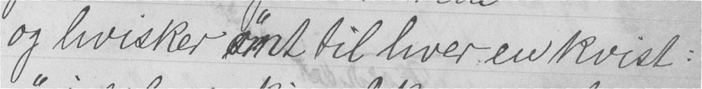og hvisker ømt til hver en kvist:
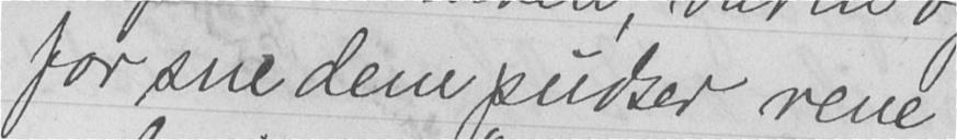for sne dem pudser rene
